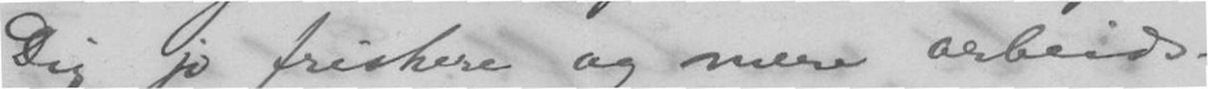Dig jo friskere og mere arbeids-
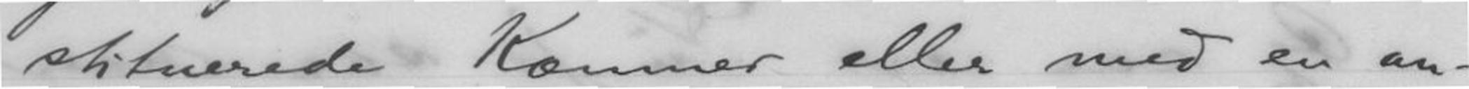stituerede Kæmner eller med en an-
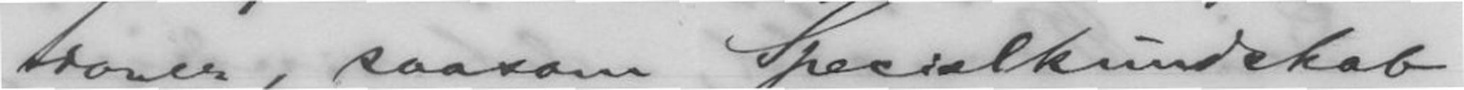tioner. saasom Specialkundskab
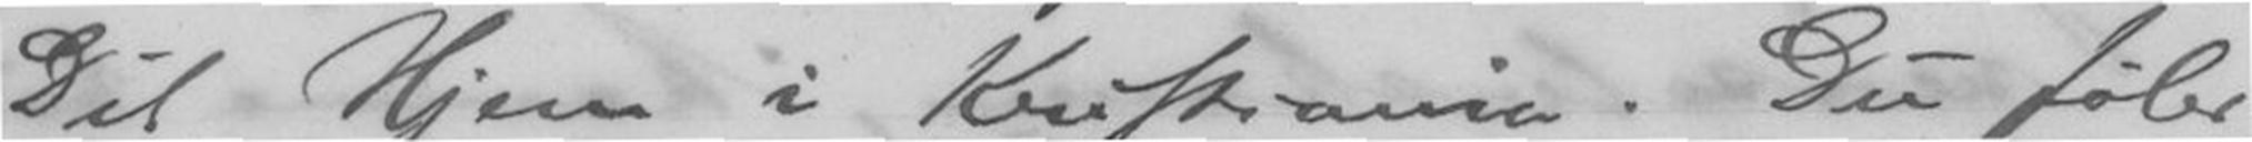Dit Hjem i Kristiania. Du føler
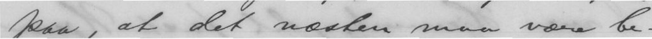paa, at det næsten maa være be-
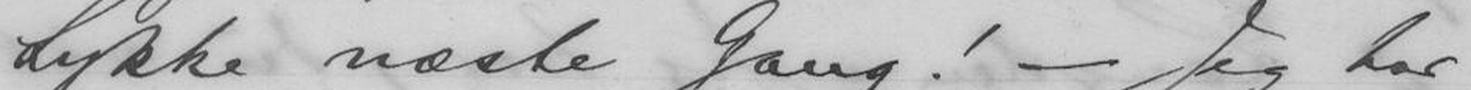Lykke næste Gang! - Jeg har
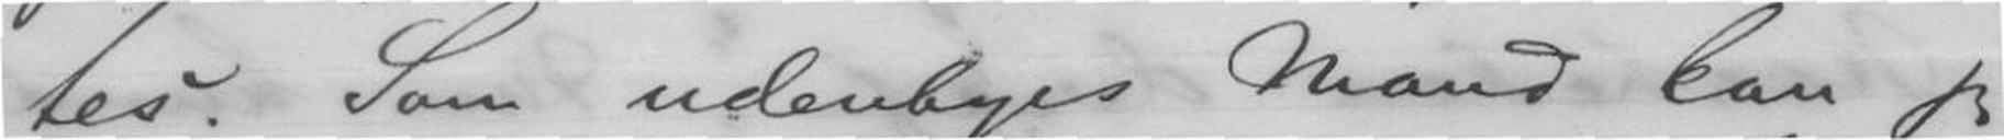tes. Som udenbyes Mand kan jeg
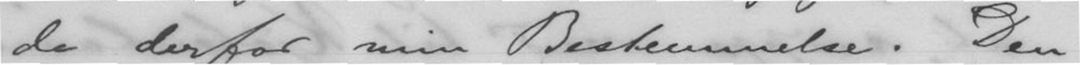de derfor min Bestemmelse. Den
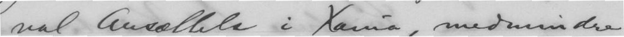nal Ansættelse i Xania, medmindre
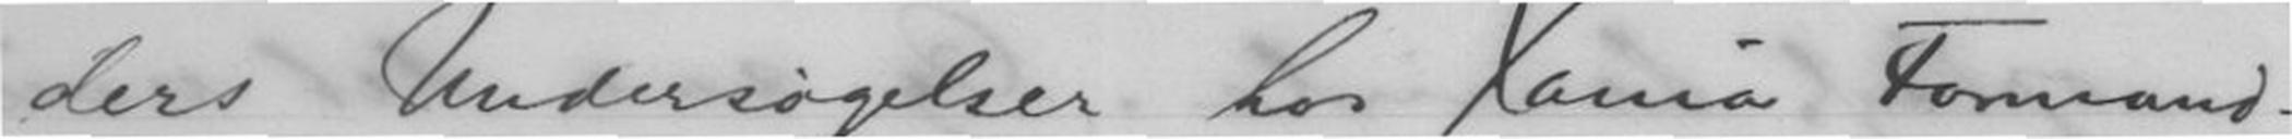ders Undersøgelser hos Xania Formand-
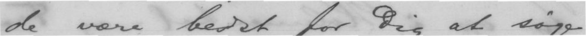de være bedst for Dig at søge
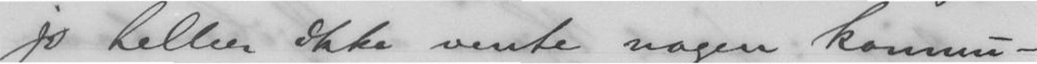jo heller ikke vente nogen kommu-
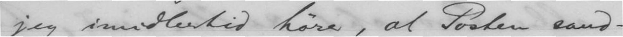jeg imidlertid høre, at Posten sand-
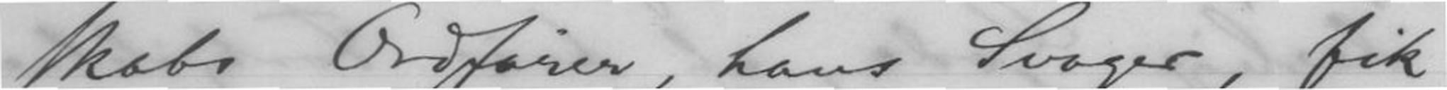skabs Ordfører, hans Svoger, fik
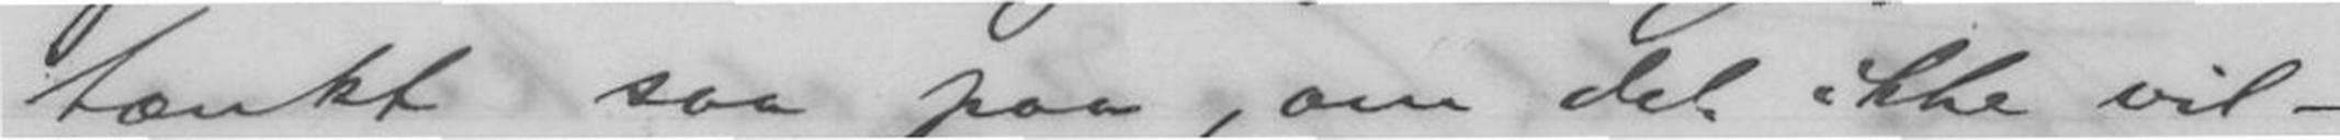tænkt saa paa, om det ikke vil-
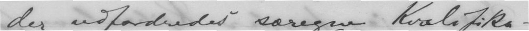der udfordres særegne Kvalifika-
